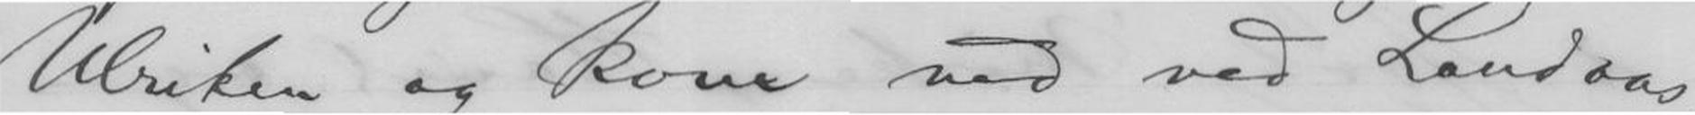Ulriken og kom ned ved Landaas,
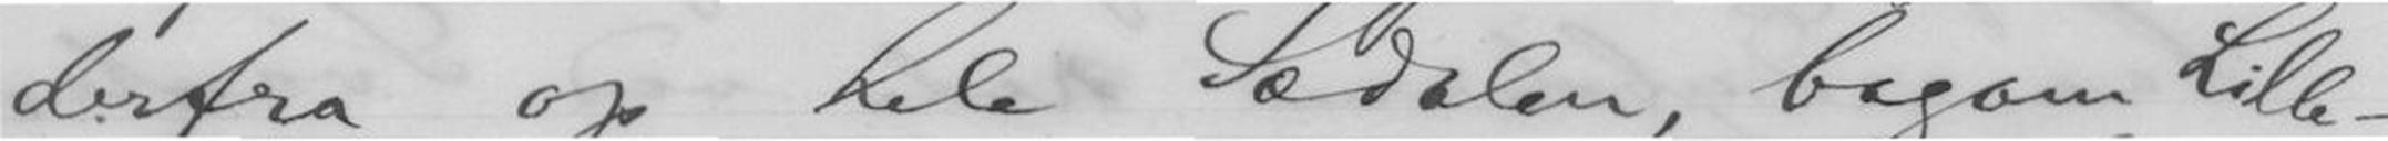derfra op hele Sædalen, bagom Lille-
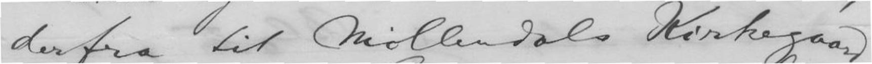derfra til Møllendals Kirkegaard
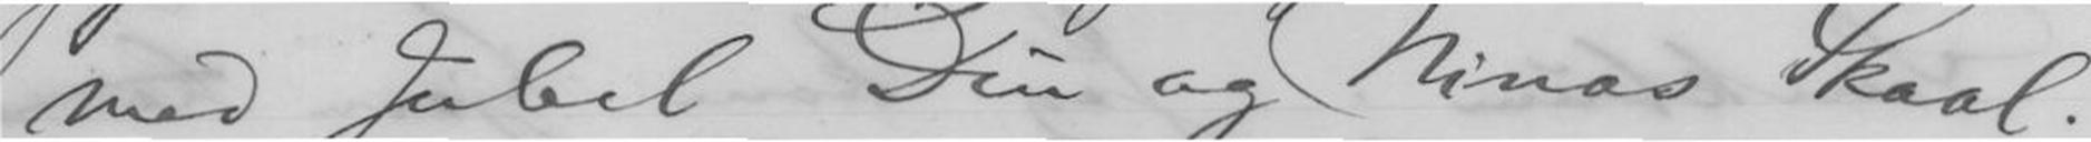med Jubel Din og Ninas Skaal.
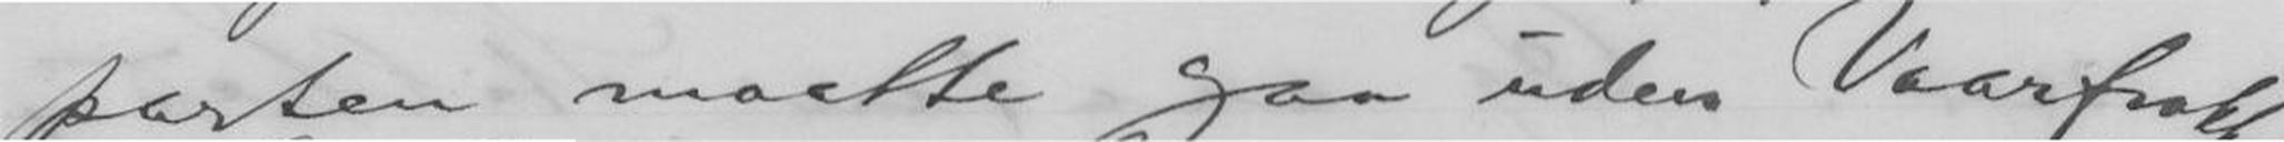parten maatte gaa uden Vaarfrakke.
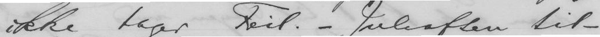ikke tager Feil. - Juleaften til-
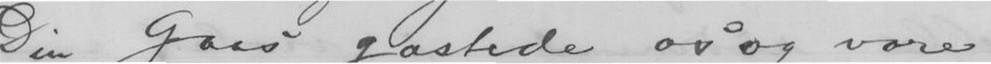Din Gaas gastede os og vore
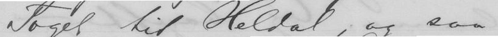Toget til Heldal, og saa
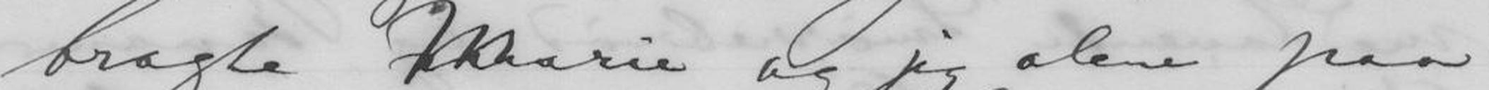bragte Marie og jeg alene paa
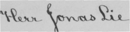Herr Jonas Lie.
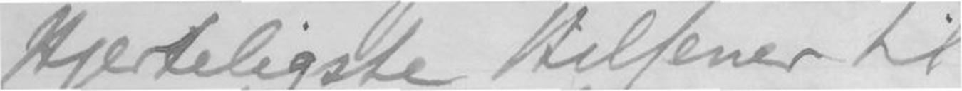Hjerteligste Hilsener til
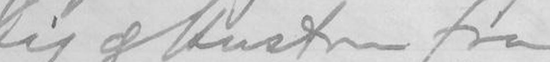Dig og Hustru fra
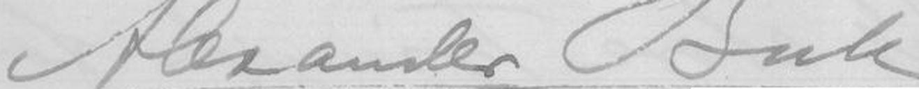Alexander Bull
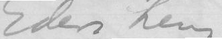Eders hengivne
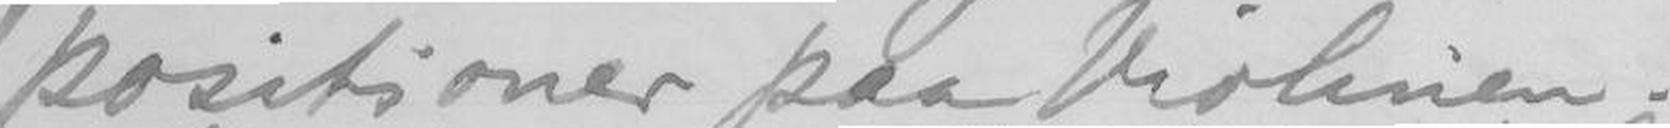positioner paa Violinen.
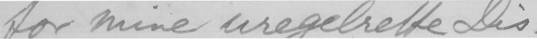for mine uregelrette Dis-
